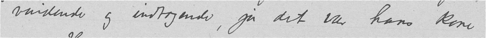vindende og indtagende, ja det var hans kone
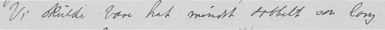Vi skulde bare hat mindst dobbelt saa lang
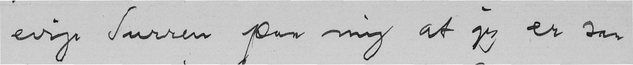evige Surren paa mig at jeg er saa
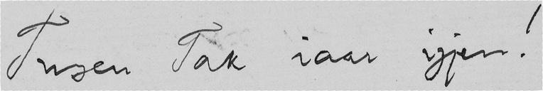Tusen Tak iaar igjen!
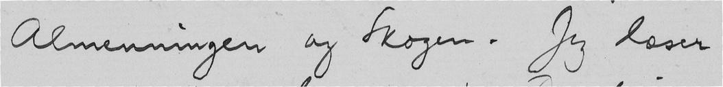Almenningen og Skogen. Jeg læser
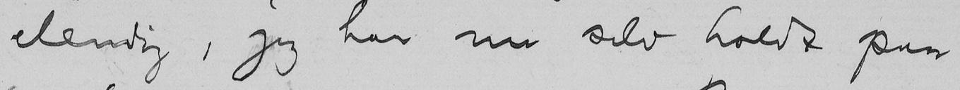elendig, jeg har nu selv holdt paa
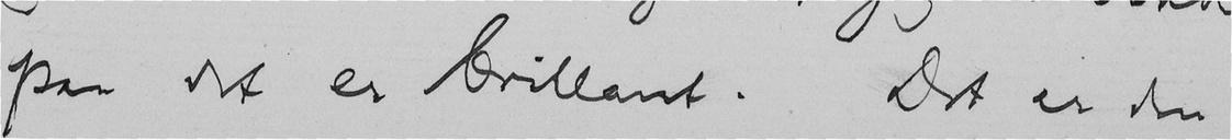paa det er brillant. Det er den
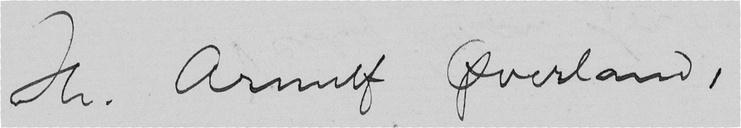Hr. Arnulf Øverland,
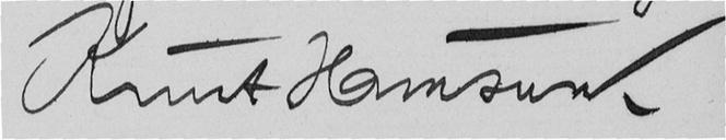Knut Hamsun.
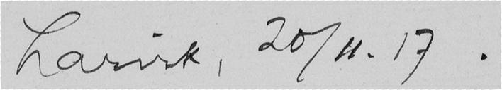Larvik, 20/11. 17.
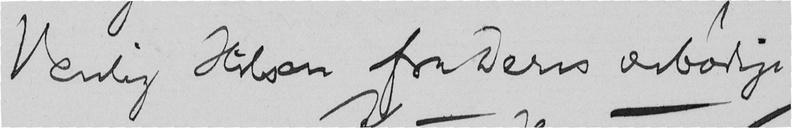Venlig Hilsen fra Deres ærbødige
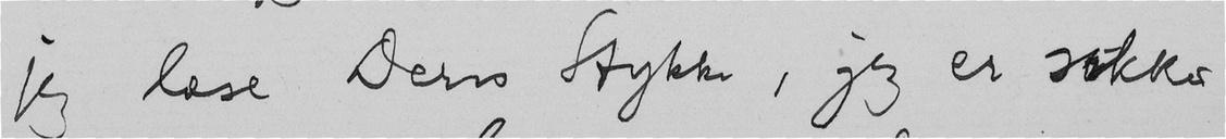jeg læse Deres Stykke, jeg er sikker
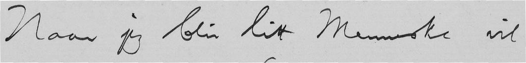Naar jeg blir litt Menneske vil
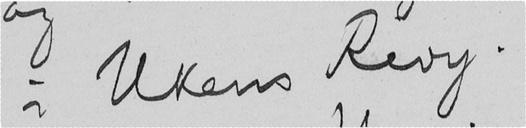i Ukens Revy.
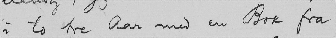i to tre Aar med en Bok fra
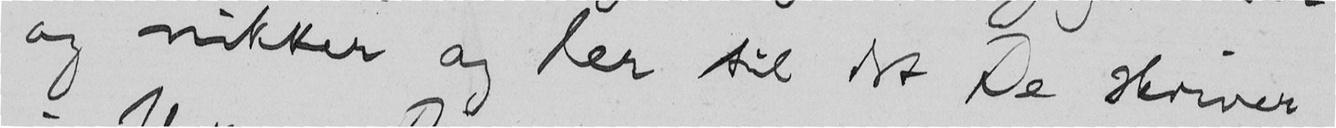og nikker og ler til det De skriver
